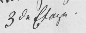3de Etage.
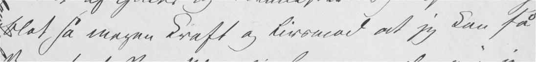blot så megen Kraft og Livsmod at jeg kan få
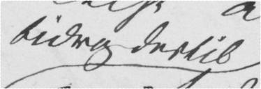bidrog dertil
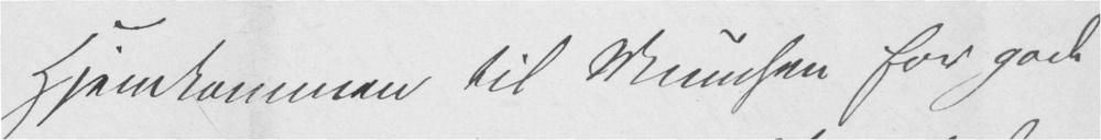Hjemkommen til München for godt
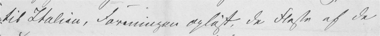til Italien, Foreningen opløst, de Fleste af de
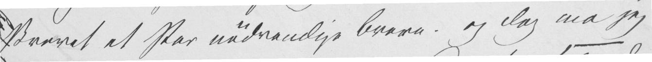skrevet et Par nødvendige Breve. og dog må jeg
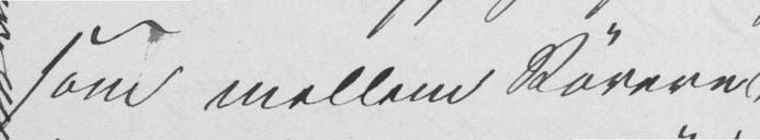som mellem Røvere
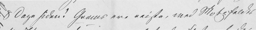8 Dage siden! Grams ere reiste, med Motzfeldts
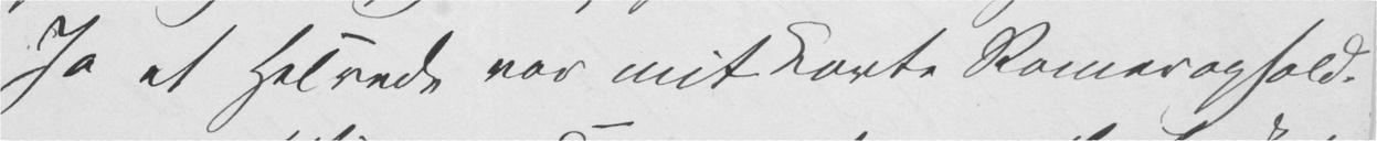Ja et Helvede var mit korte Romerophold.
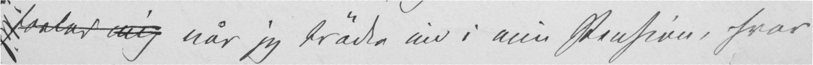forlod mig når jeg trådte ind i min Pension, hvor
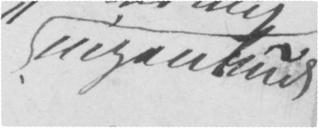ingenlunde
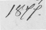1877.
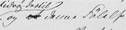og at denne Følelse
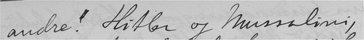andre! Hitler og Mussolini,
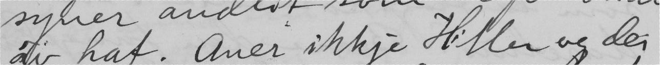av hat. Aner ikkje Hitler og dei
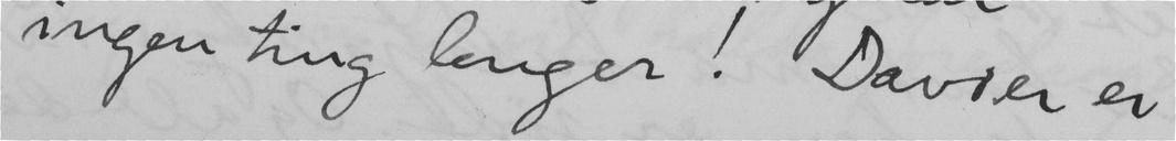ingen ting lenger! Davier er
